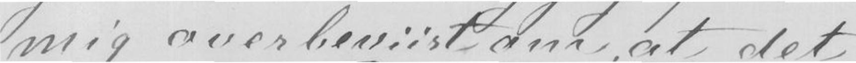mig overbeviist om, at det
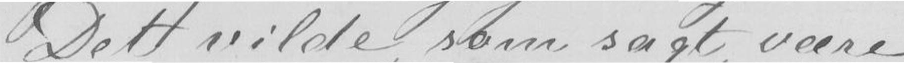Det vilde, som sagt, være
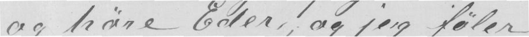og høre Eder; og jeg føler
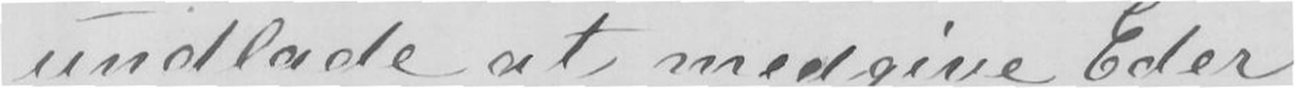undlade at medgive Eder
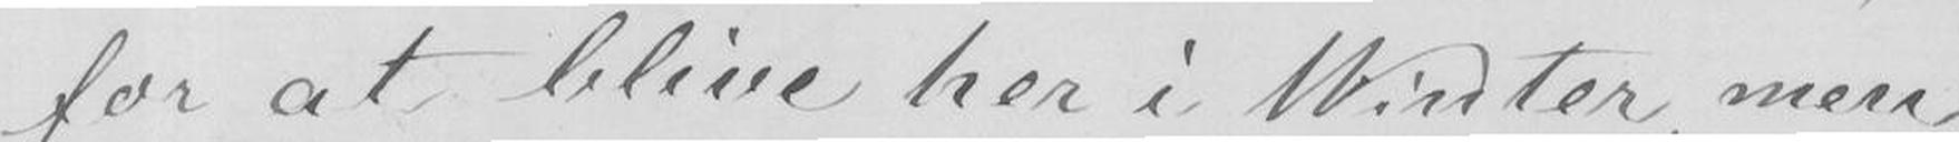for at blive her i Winter, men
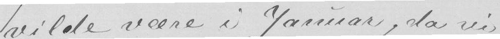vilde være i Januar, da vi
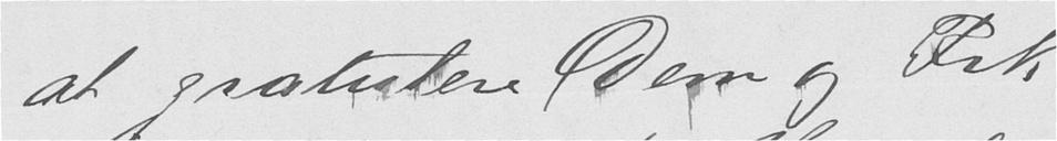at gratulere Dem og Frk
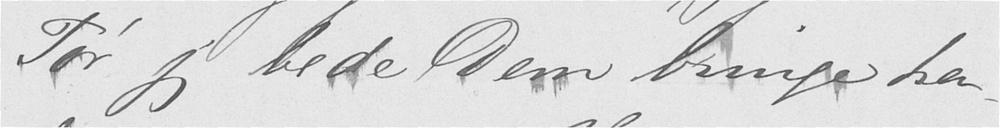Tør jeg bede Dem bringe hen-
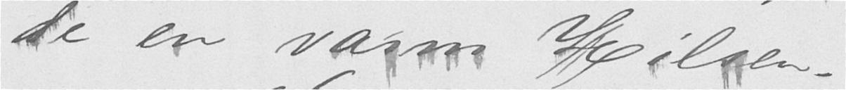de en varm Hilsen.
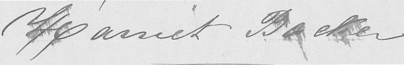Harriet Backer
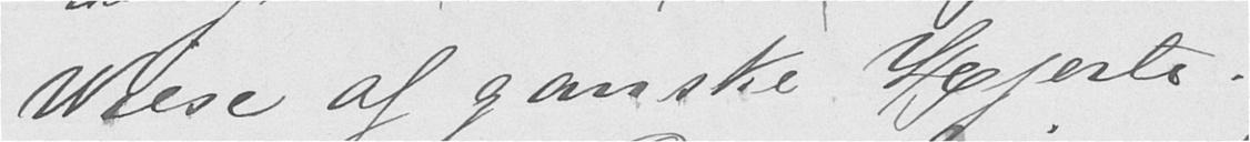Wiese af ganske Hjerte.
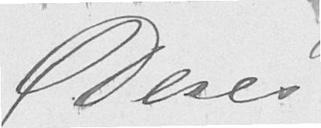Deres
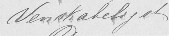Venskabeligst
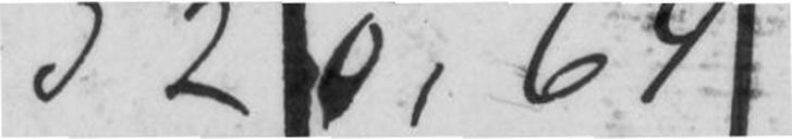320,64
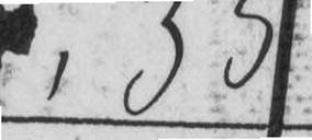,35
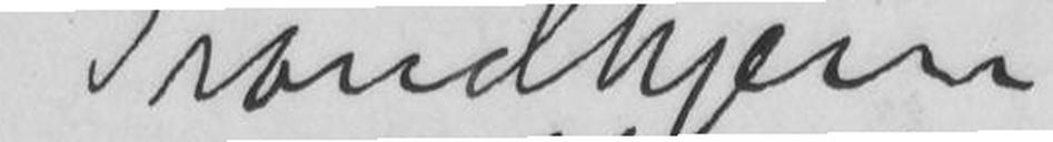Trondhjem
